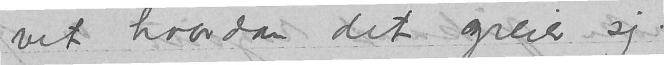vet hvordan det greier sig.
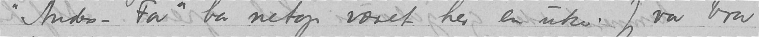«Anders-Far» har netop været her en uke. Jeg var bra
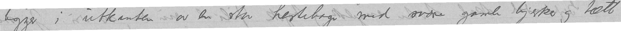ligger i utkanten av en stor hestehage med svære gamle bjerker og tætt
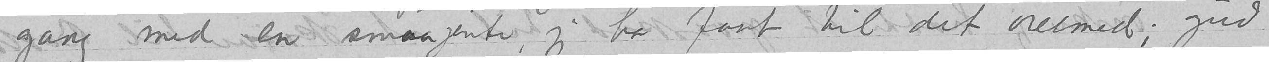gang med en smaajente, jeg har faat til det øiemed; gud
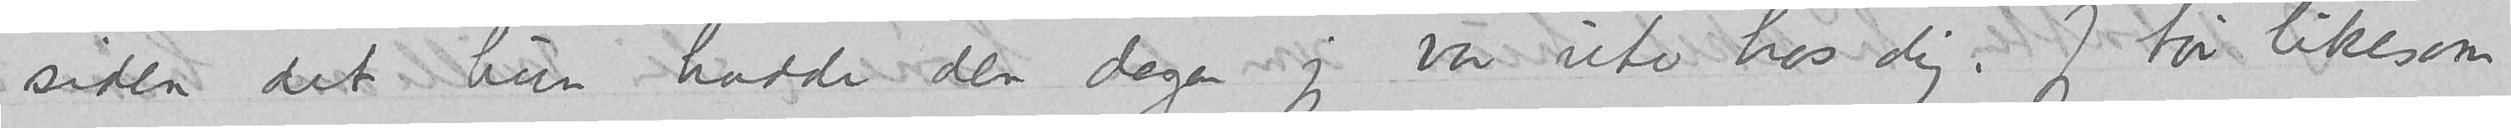siden det hun hadde den dagen jeg var ute hos dig. Jeg tør likesom
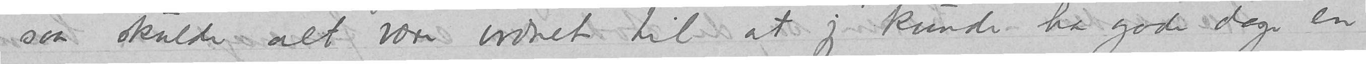saa skulde alt være ordnet til at jeg kunde ha gode dage en
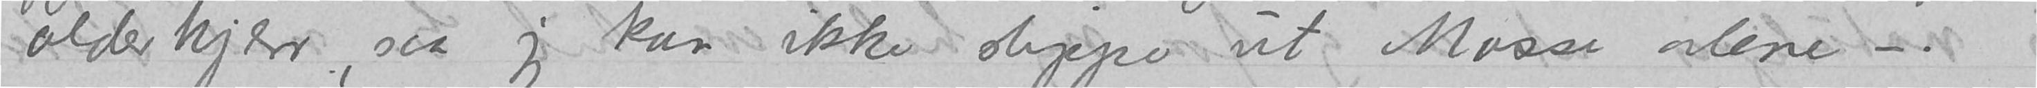olderkjerr, saa jeg kan ikke slippe ut Mosse alene –.
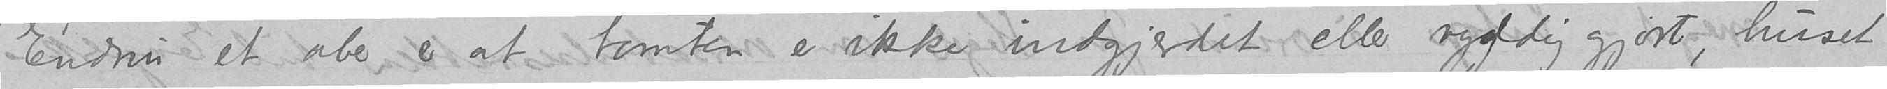Endnu et aber er at tomten er ikke indgjerdet eller ryddig gjort, huset
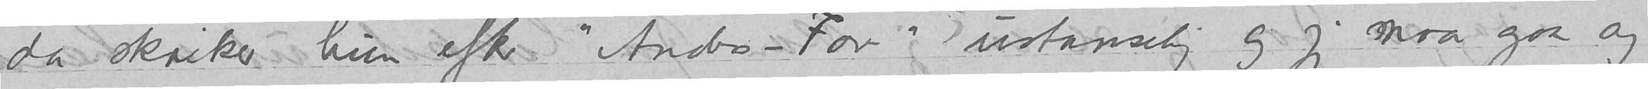da skriker hun efter «Anders-Far» ustanselig og jeg maa gaa og
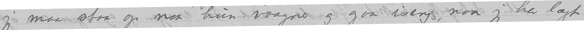jeg maa staa op naar hun vaagner og gaa iseng, naar jeg har lagt
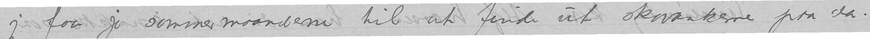jeg faar jo sommermaanderne til at finde ut skavankerne paa da.
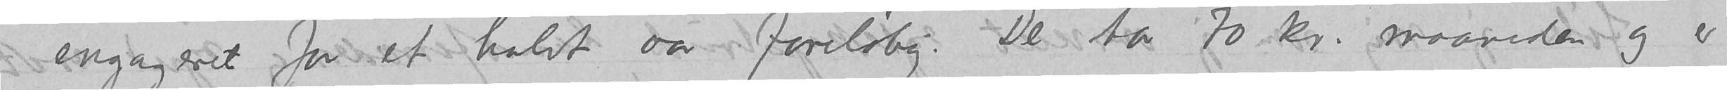engageret for et halvt aar foreløbig. De tar 70 kr. maaneden og er
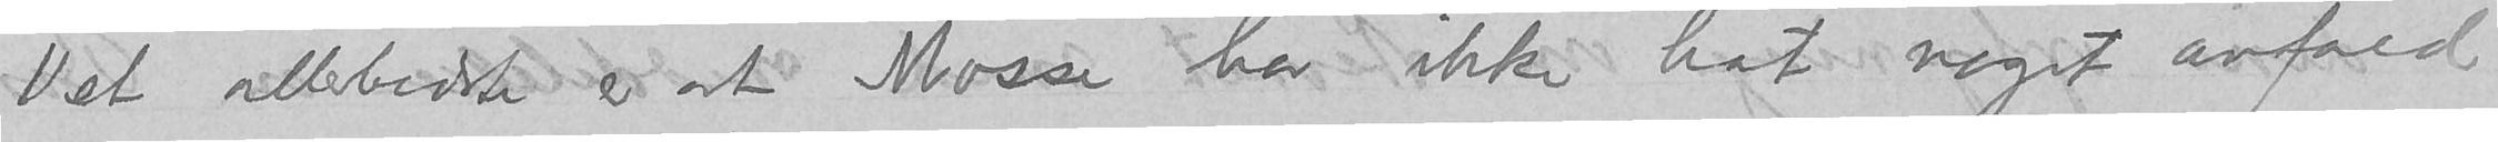Det allerbedste er at Mosse har ikke hat noget anfald
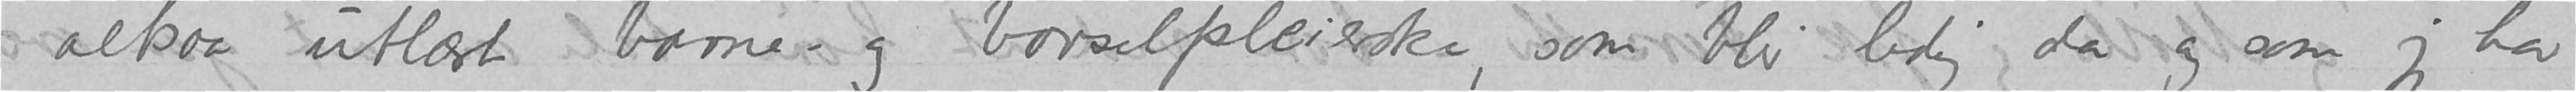altsaa utlært barne- og barselpleierske, som blir ledig da og som jeg har
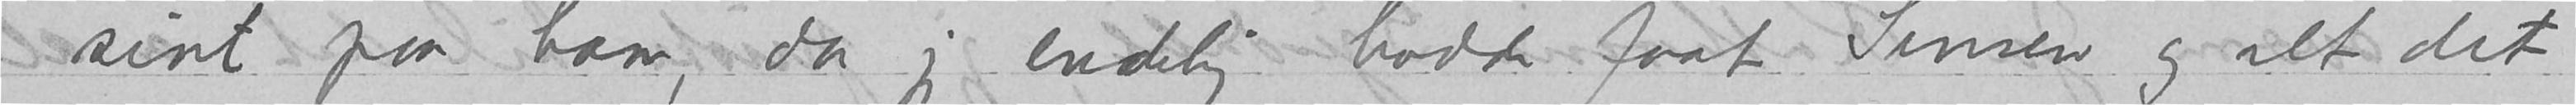sint paa ham, da jeg endelig hadde faat Sinsen og alt det
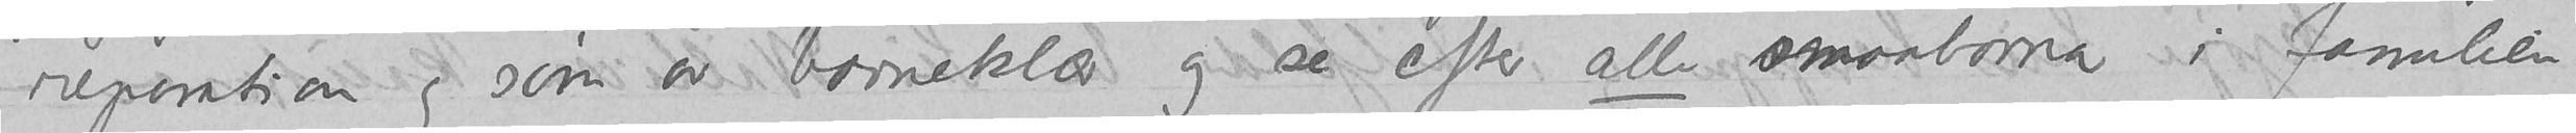reperation og søm av barneklær og se efter alle smaabarna i
